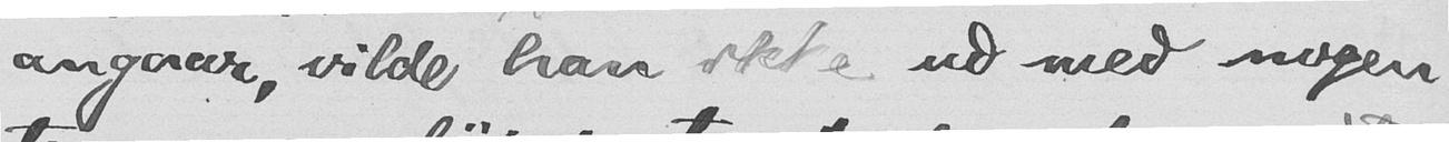angaar, vilde han ikke ud med nogen
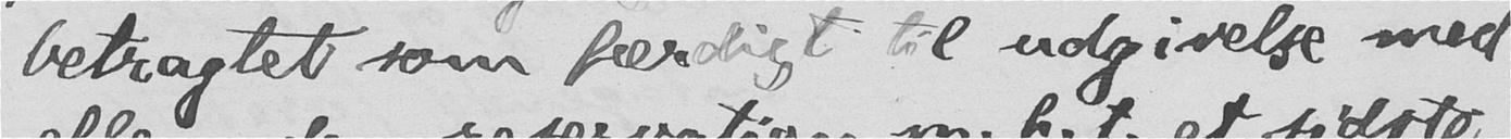betragtet som færdigt til udgivelse med
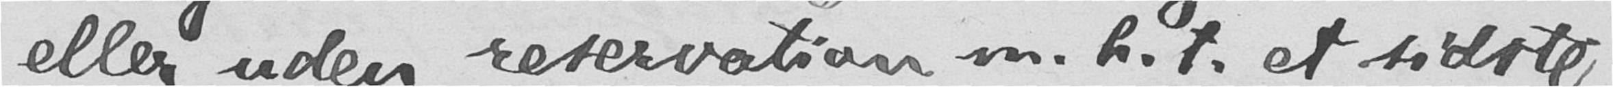eller uden reservation m. h. t. et sidste
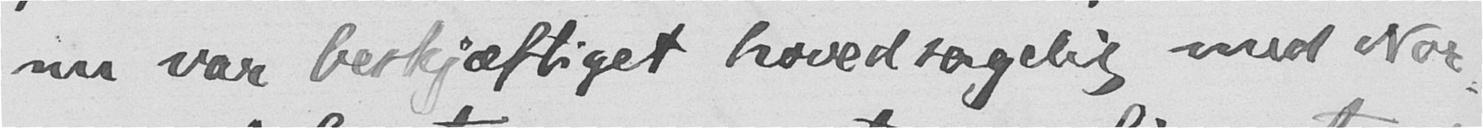nu var beskjæftiget hovedsagelig med Nor-
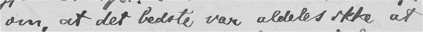om, at det bedste var aldeles ikke at
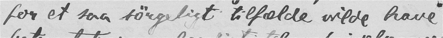for et saa sørgeligt tilfælde vilde have
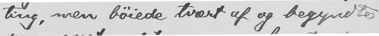ting, men bøiede tvært af og begyndte
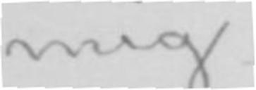mig
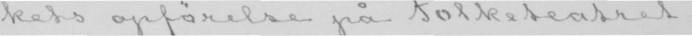kets opførelse på Folketeatret.
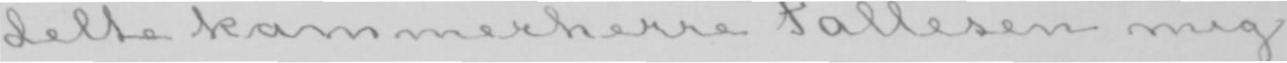delte kammerherre Fallesen mig
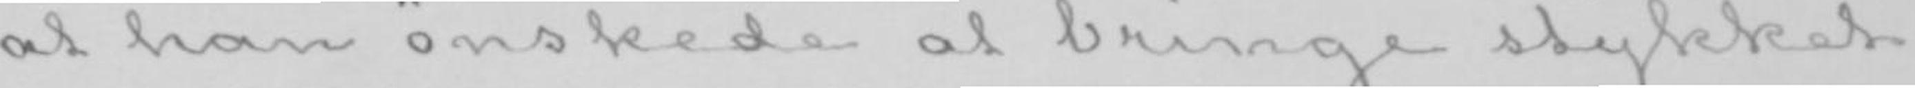at han ønskede at bringe stykket
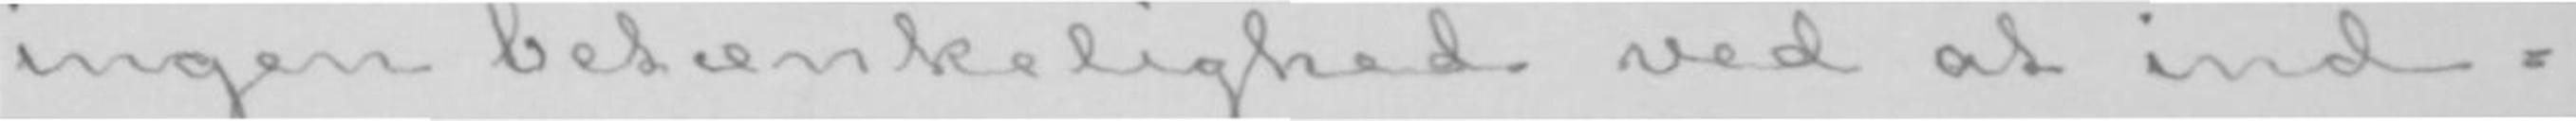ingen betænkelighed ved at ind-
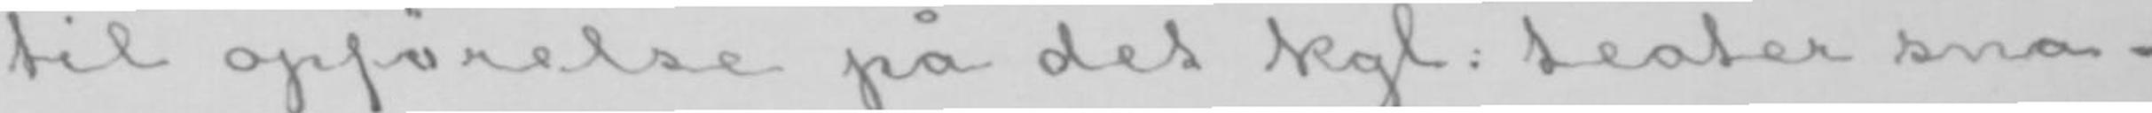til opførelse på det kgl: teater sna-
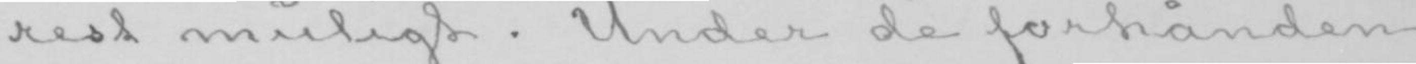rest muligt. Under de forhånden
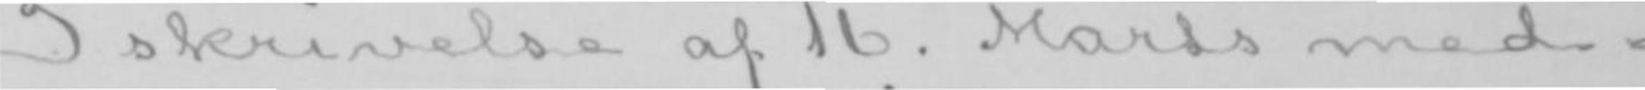I skrivelse af 16. Marts med-
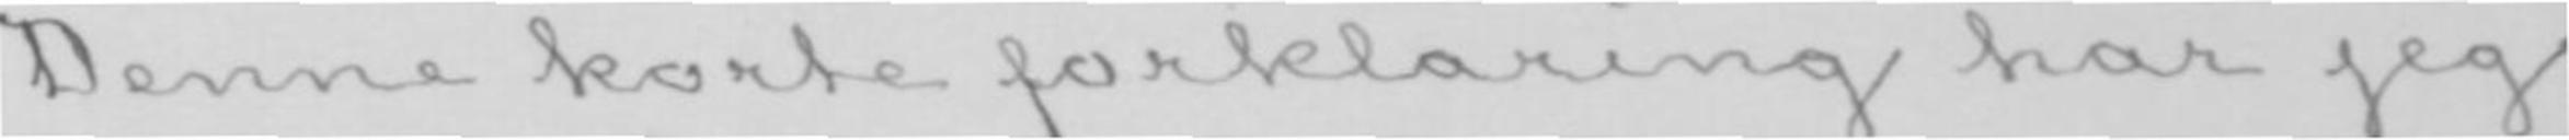Denne korte forklaring har jeg
